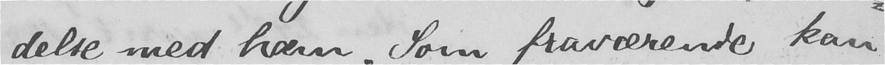delse med ham. Som fraværende kan
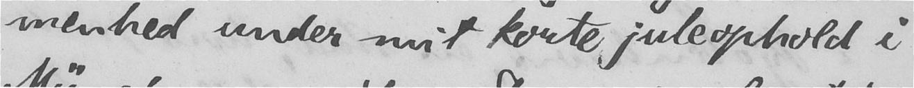menhed under mit korte juleophold i
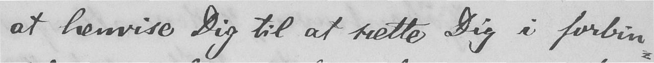at henvise Dig til at sætte Dig i forbin-
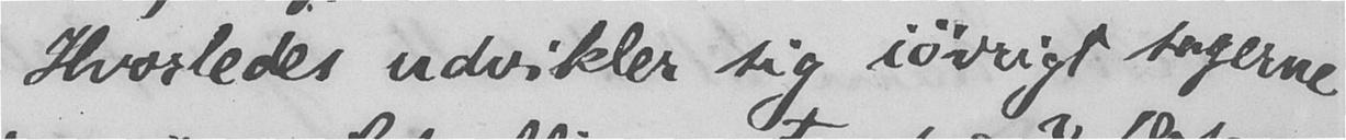Hvorledes udvikler sig iøvrigt sagerne
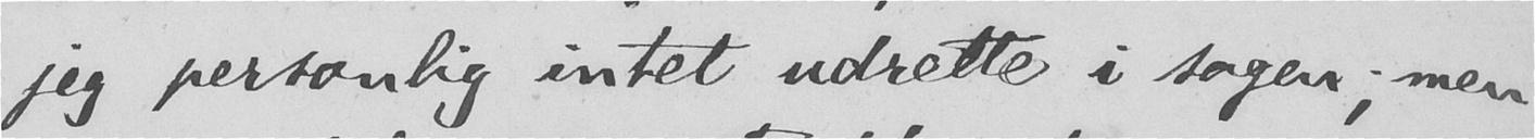jeg personlig intet udrette i sagen; men
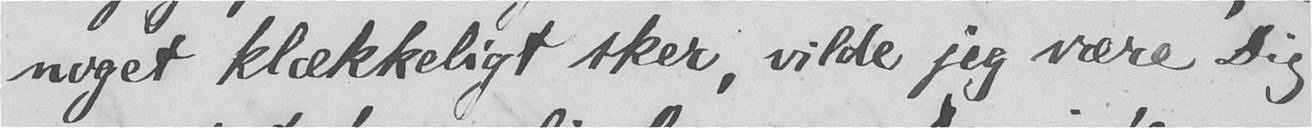noget klækkeligt sker, vilde jeg være Dig
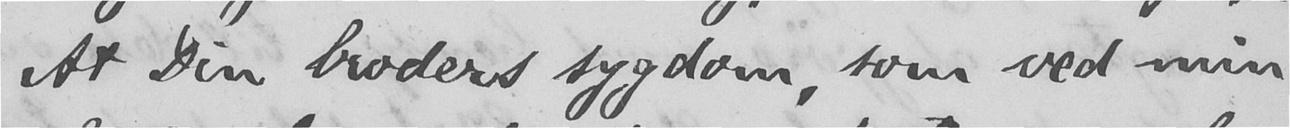At Din broders sygdom, som ved min
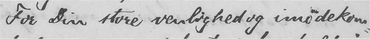For Din store venlighed og imødekom-
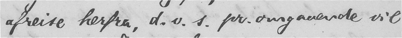afreise herfra, d. v. s. pr. omgaaende vil
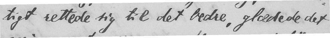tigt rettede sig til det bedre, glædede det
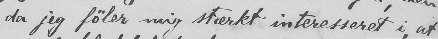da jeg føler mig stærkt interesseret i, at
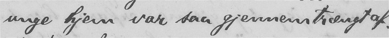unge hjem var saa gjennemtrængt af.
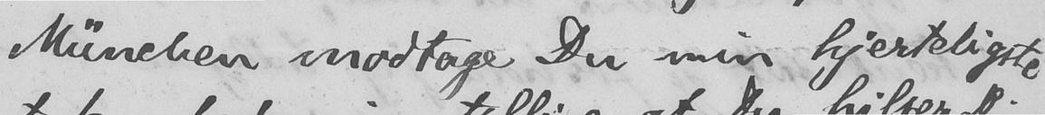München modtage Du min hjerteligste
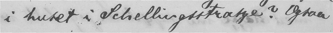i huset i Schellingsstrasse? Ogsaa
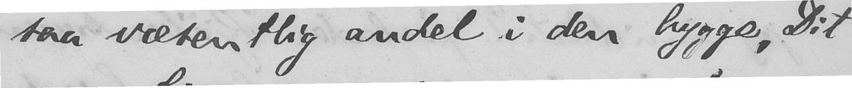saa væsentlig andel i den hygge, Dit
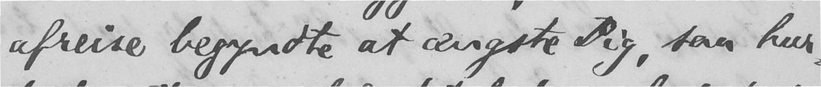afreise begyndte at ængste Dig, saa hur-
